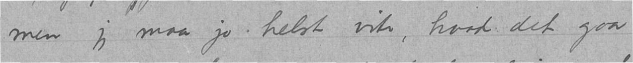men jeg maa jo helst vite, hvad det gaar
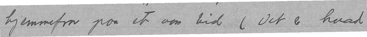hjemmefra paa et aars tid (Det er hvad
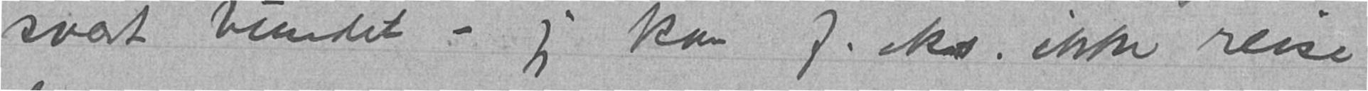svært bundet – jeg kan f.eks. ikke reise
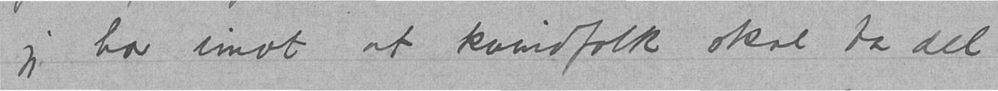jeg har imot at kvindfolk skal ta del
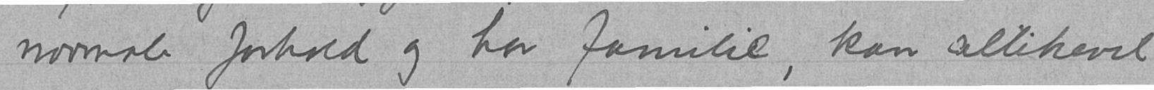normale forhold og har familie, kan allikevel
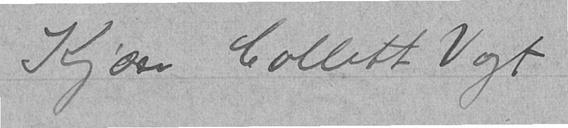Kjære Collett Vogt
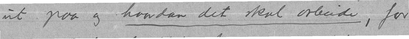ut paa og hvordan det skal arbeides, for
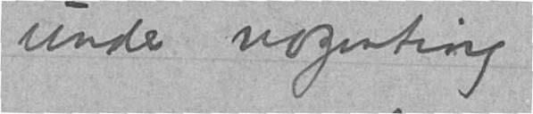under nogenting?
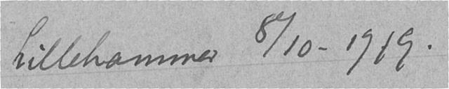Lillehammer 8/10-1919.
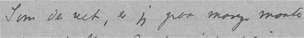Som De vet, er jeg paa mange maater
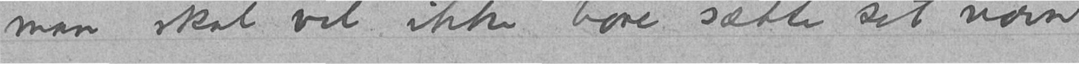man skal vel ikke bare sætte sit navn
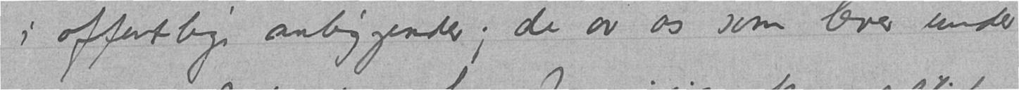i offentlige anliggender; de av os som lever under
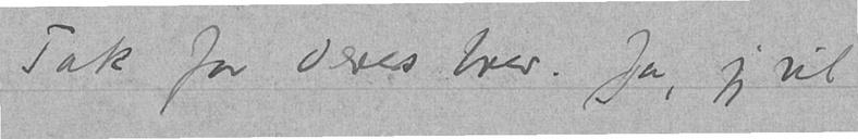Tak for Deres brev. Ja, jeg vil
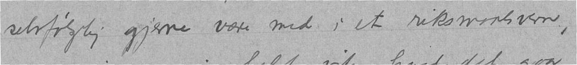selvfølgelig gjerne være med i et riksmaalsvern,
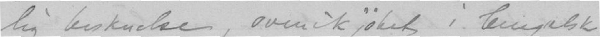lig beskuelse, ovenikjøbet i bengalsk
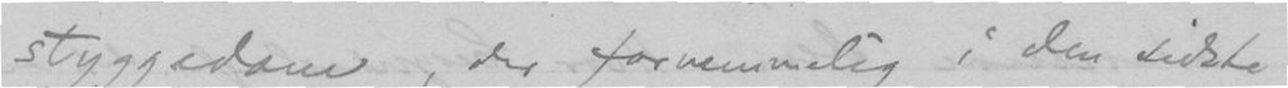styggedom, der forvemmelig i den sidste
